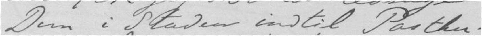Dem i Staden intil Posthu-
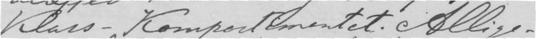Klass-Kompartementet. Allige-
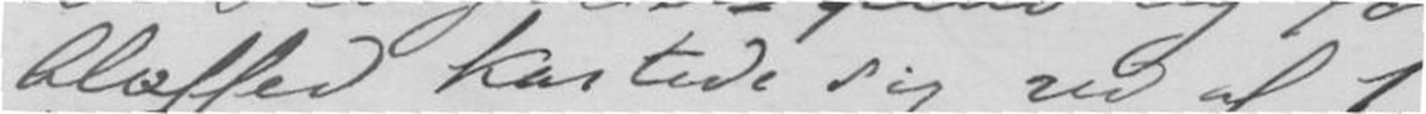bløffed kastede sig ud af 1e
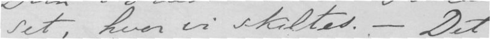set, hvor vi skiltes.- Det
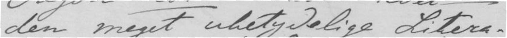den meget ubetydelige Litera-
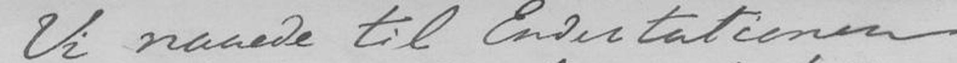- Vi naaede til Endestationen
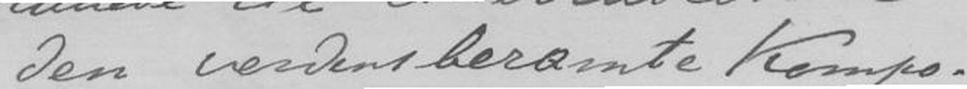den verdensberømte Kompo-
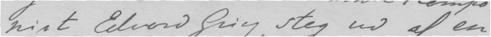nist Edbvard Grieg steg ud af en
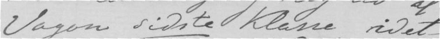Vagon siste Klasse idet
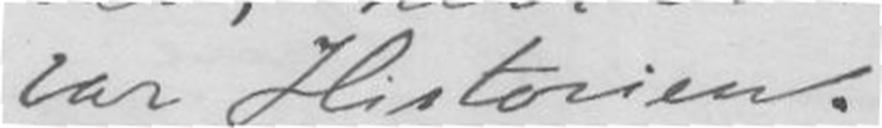var Historien.
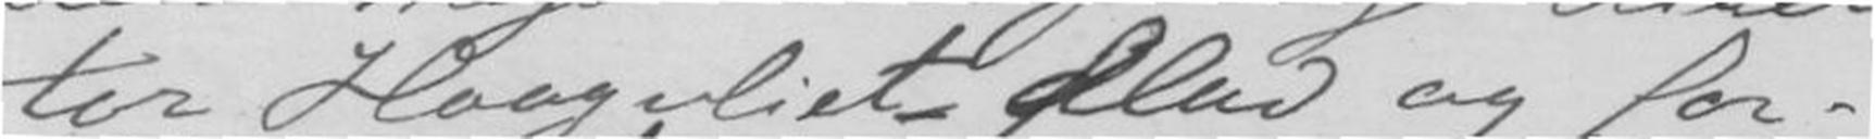tor Hoogenlict, flad og for-
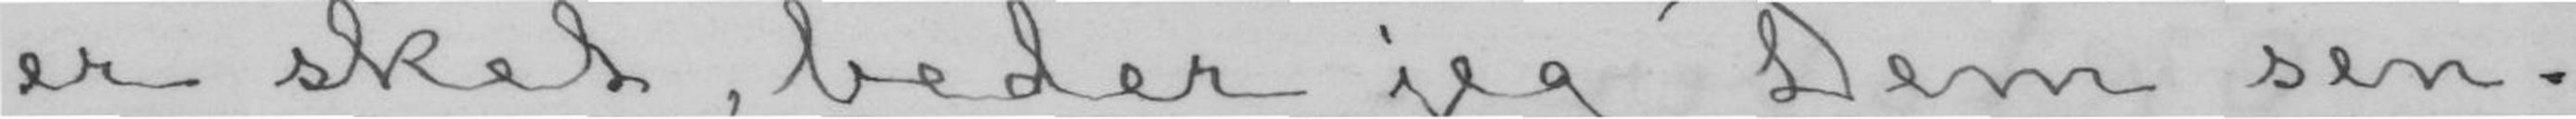er sket, beder jeg Dem sen-
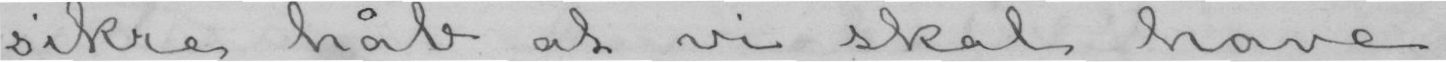sikre håb at vi skal have
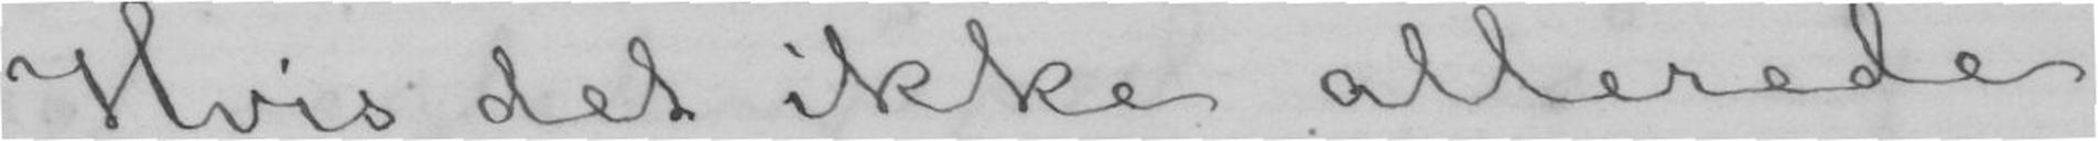Hvis det ikke allerede
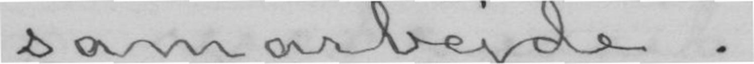samarbejde.
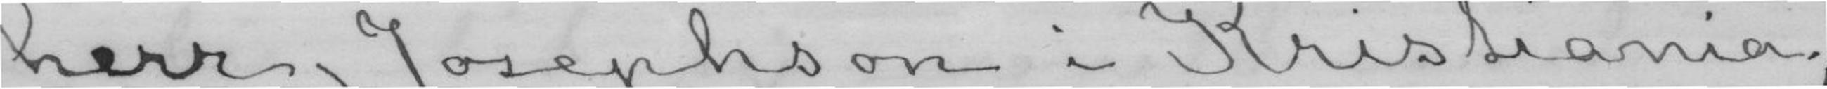herr Josephson i Kristiania;
